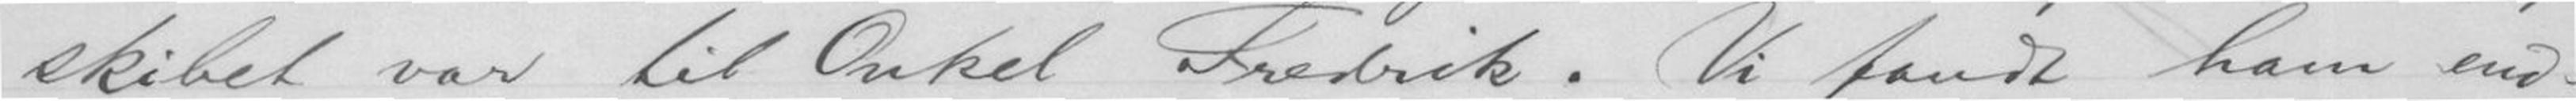skibet var til Onkel Fredrik. Vi fandt ham end-
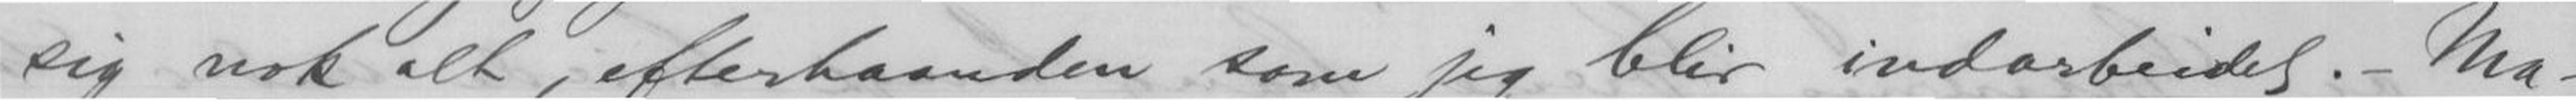sig nok alt, efterhaanden som jeg blir indarbeidet. - Ma-
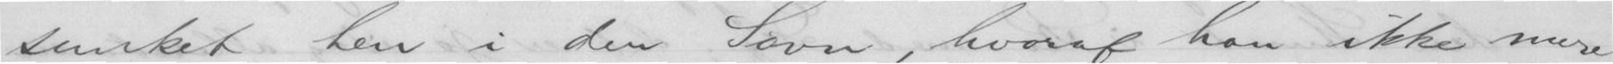sunket hen i den Søvn, hvoraf han ikke mere
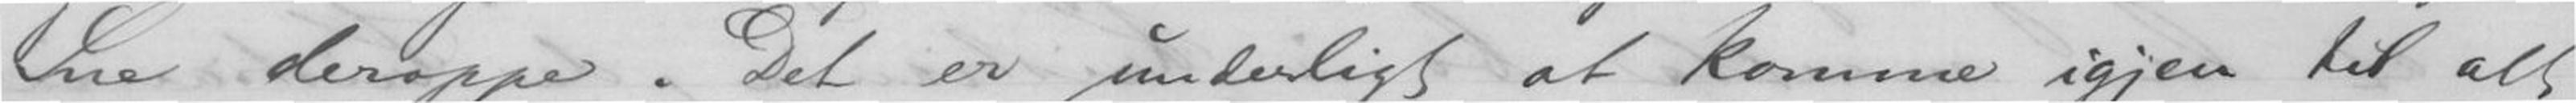Sne deroppe. Det er underligt at komme igjen til alt
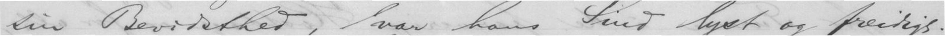sin Bevidsthed, var hans Sind lyst og freidigt.
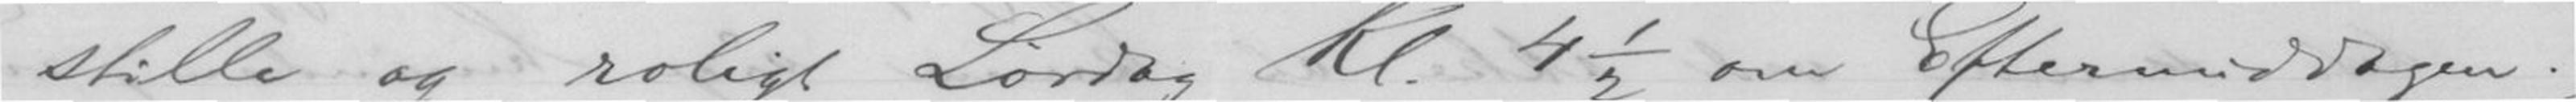stille og roligt Lørdag Kl. 4½ om Eftermiddagen.
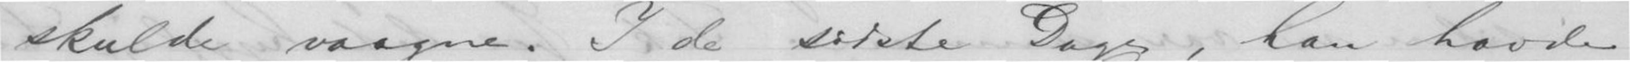skulde vaagne. I de sidste Dage, han havde
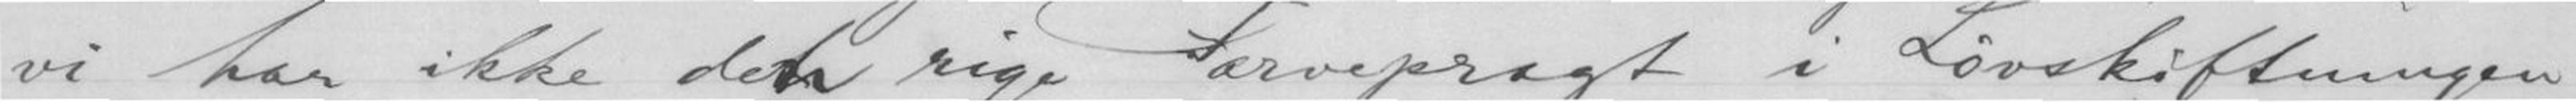vi har ikke den rige Farvepragt i Løvskiftningen
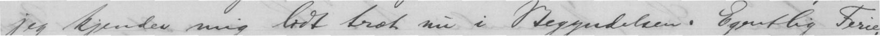jeg kjender mig lidt træt nu i Begyndelsen. Egentlig Ferie,
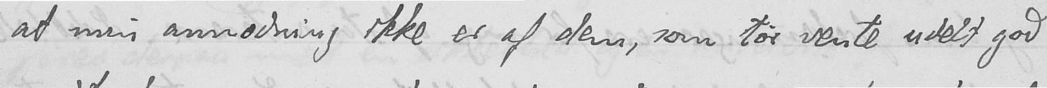at min anmodning ikke er af dem, som tør vente udelt god
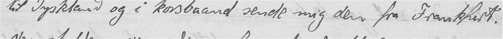til Tyskland og i korsbaand sende mig den fra Frankfurt.
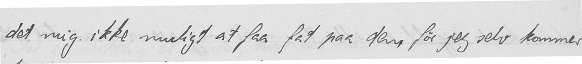det mig ikke muligt at faa fat paa den før jeg selv kommer
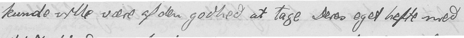kunde ville være af den godhed at tage Deres eget hefte med
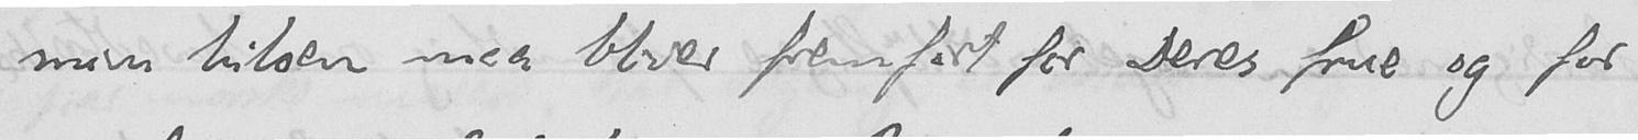min hilsen maa blive fremført for Deres frue og for
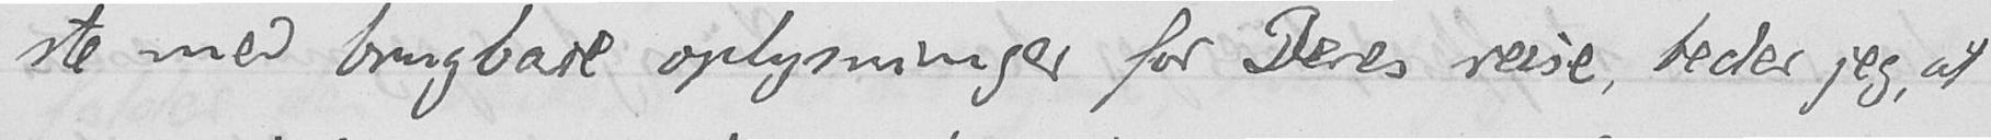ste med brugbare oplysninger for Deres reise, beder jeg, at
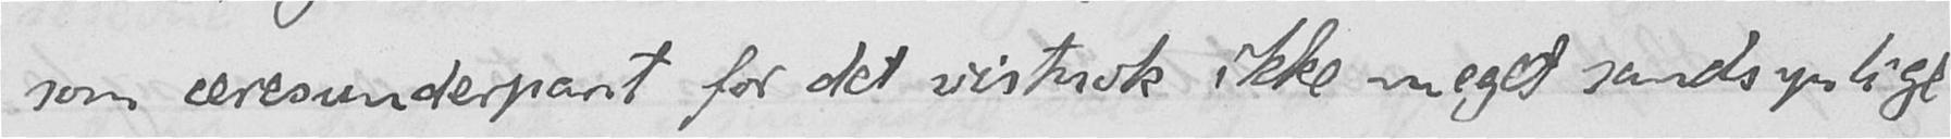som æresunderpant for det vistnok ikke meget sandsynlige
